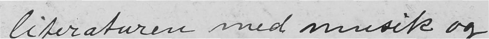literaturen med musik og
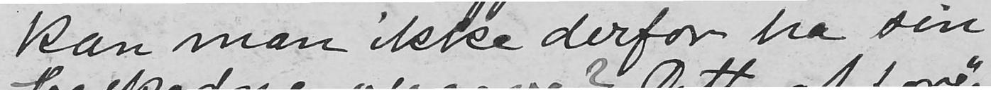kan man ikke derfor ha sin
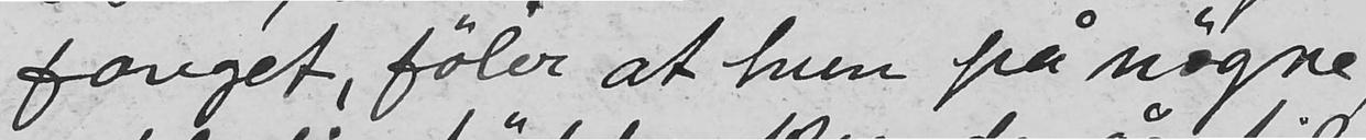fanget, føler at hun på nøgne
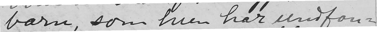barn som hun har undfan-
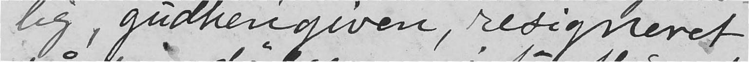lig gudhengiven resigneret
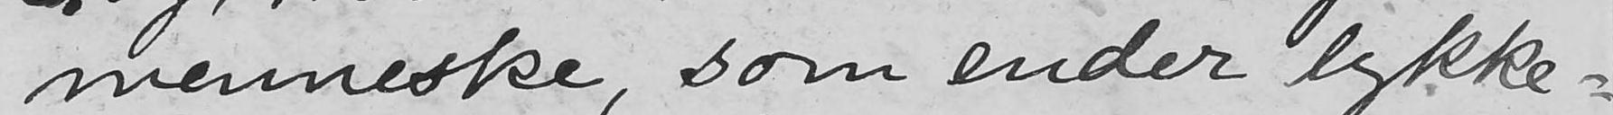menneske, som ender lykke-
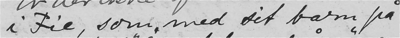i Fie, som med sit barn på
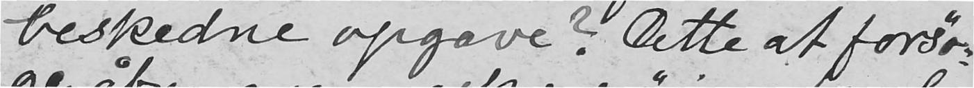beskedne opgave? Dette af forsø-
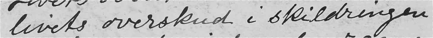livets overskud i skildringen
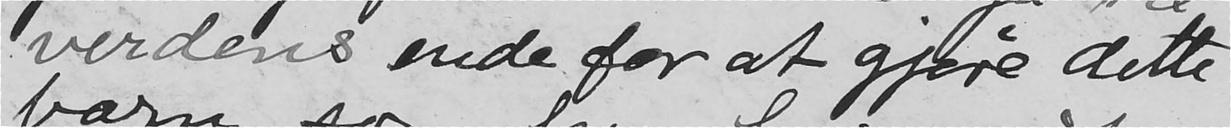verdens ende for at gjøre dette
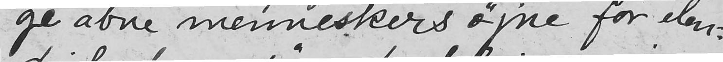ge åbne menneskers øjne for elen-
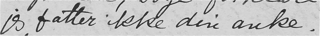jeg fatter ikke din anke.
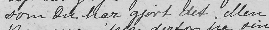som Du har gjort det. Men
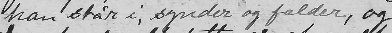han står i, synder og falder, og
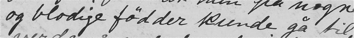og blodige fødder kunde gå til
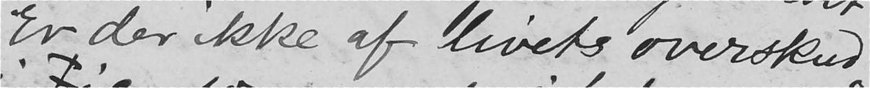Er der ikke af livets overskud
